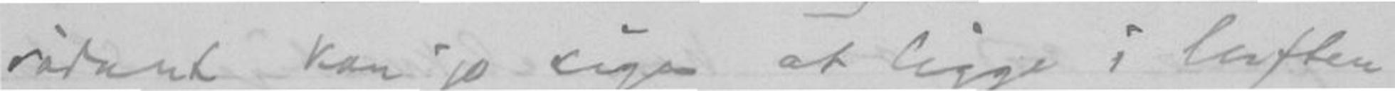sådant kan jo siges at ligge i luften
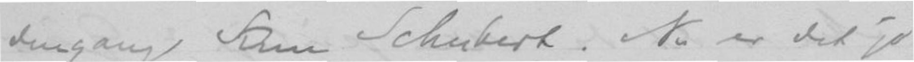dengang kun Schubert. Nu er det jo
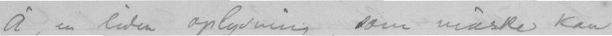Å, en liden oplysning, som måske kan
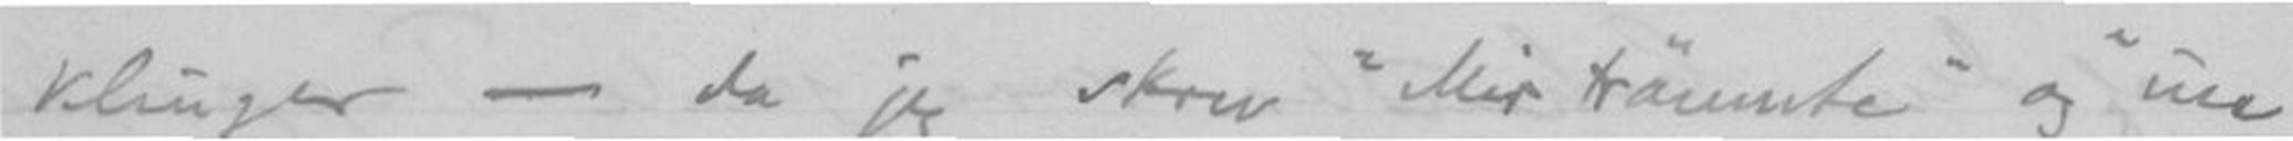klinger - da jeg skrev "Mir träumte" og "ins
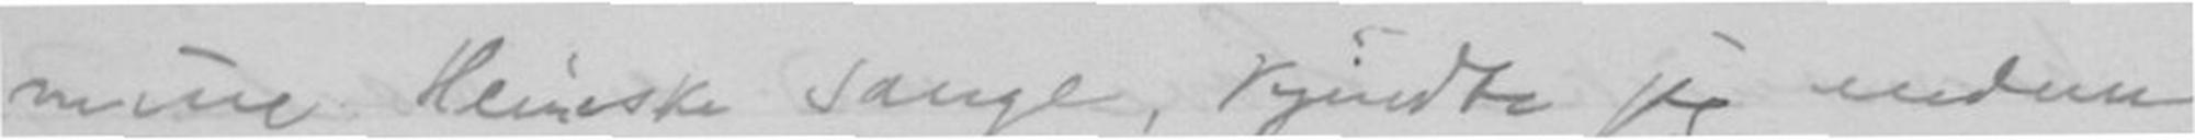mine Heineske Sange, kjendte jeg endnu
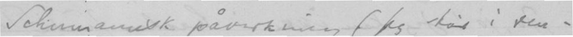Schumannsk påvirkning (jeg står i ven-
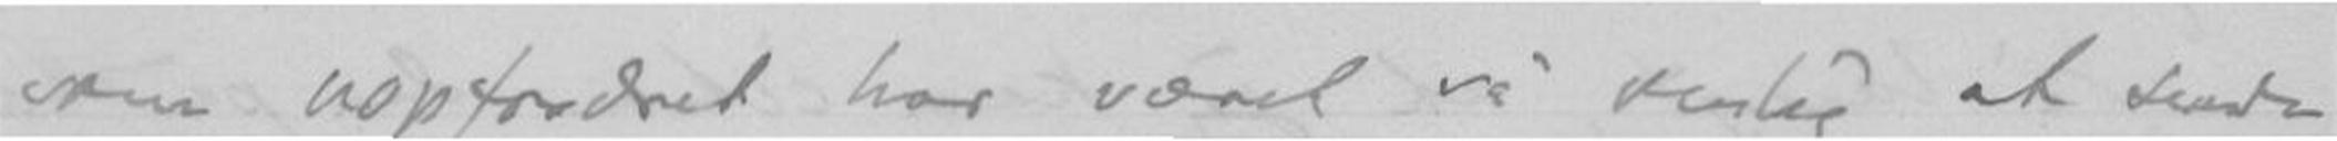som uopfordret har været så venlig at sende
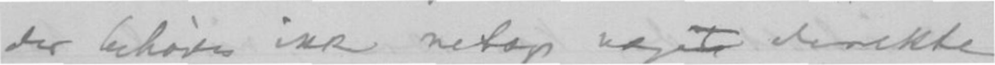der behøves ikke netop noget direkte
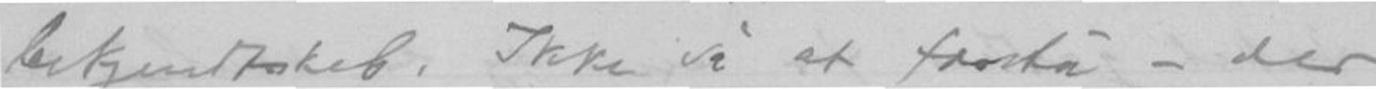bekjendskab. Ikke så at forstå - der
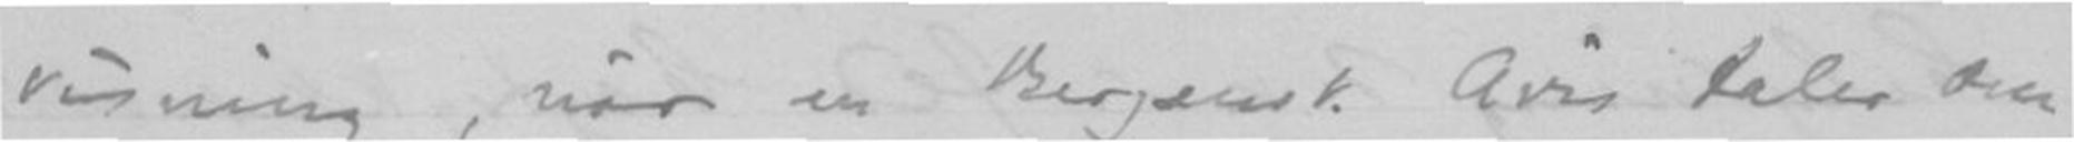visning, når en Bergens Avis taler om
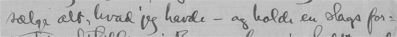sælge alt, hvad jeg havde - og holde en slags for-
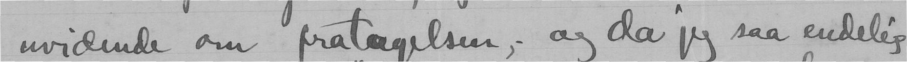uvidende om fratagelsen, - og da jeg saa endelig
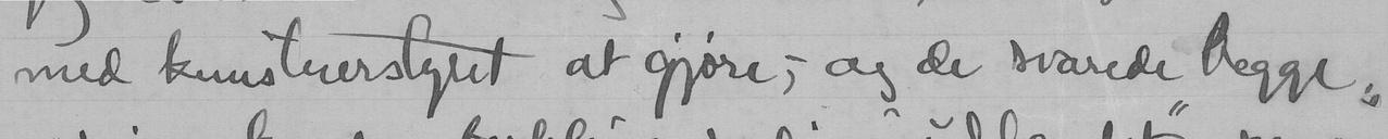med kunstnerstyret at gjøre, - og de svarede begge:
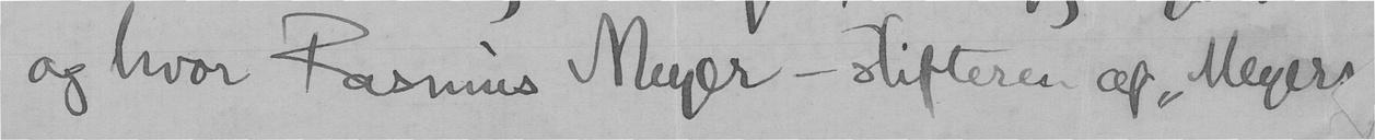og hvor Rasmus Meyer - stifteren af "Meyers
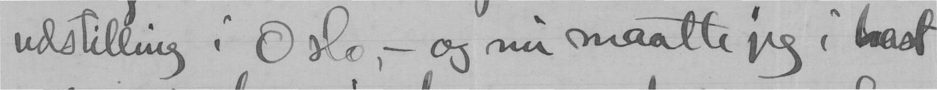udstilling i Oslo, - og nu maatte jeg i hast
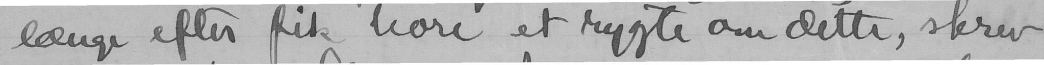længe efter fik høre et rygte om dette, skrev
